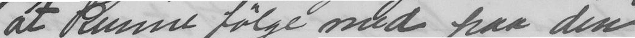at kunne følge med paa den
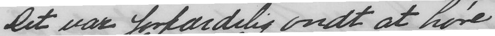Det var forfærdelig ondt at høre,
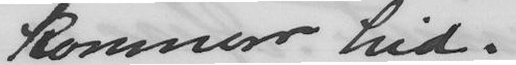kommer hid.
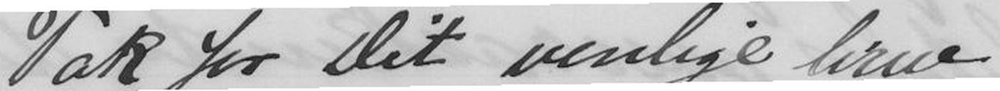Tak for Dit venlige brev
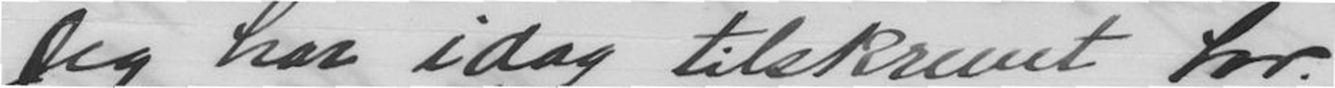Jeg har idag tilskrevet hr.
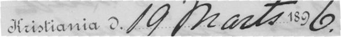Kristiania d. 19 Marts 1896.
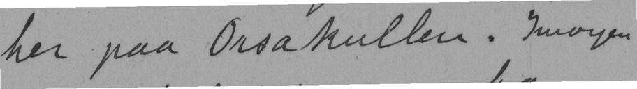her paa Orsakullen. Imorgen
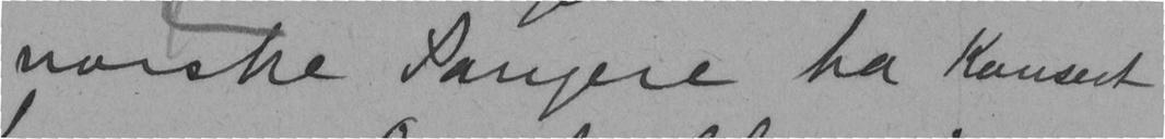norske Sangere ha Konsert
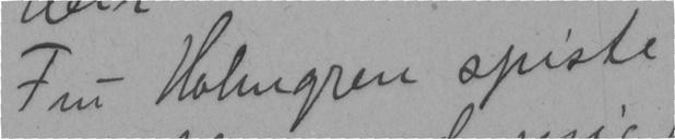Fru Holmgren spiste
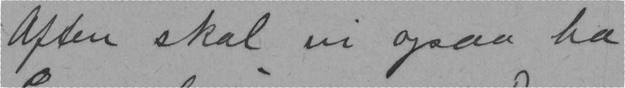Aften skal vi ogsaa ha
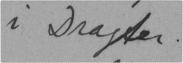i Dragter.
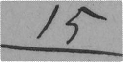15
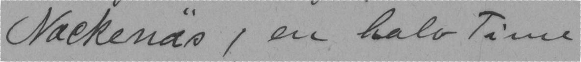Nackenäs, en halv Time
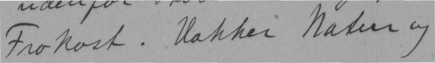Frokost. Vakker Natur og
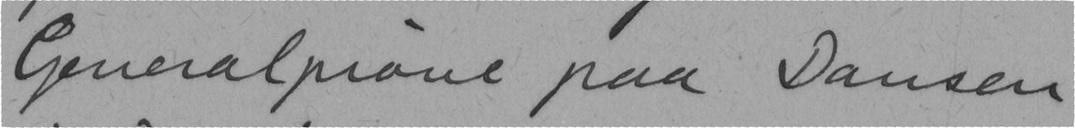Generalprøve paa Dansen
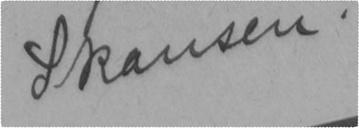Skansen.
